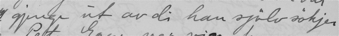gjenge ut av di han sjölv sökjer
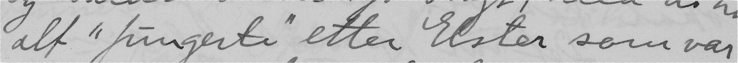alt "fungerte" etter Elster som var
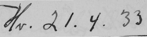Hv. 21.4.33
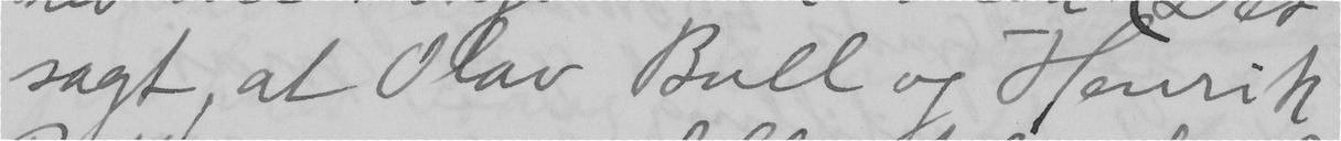sagt at Olav Bull og Henrik
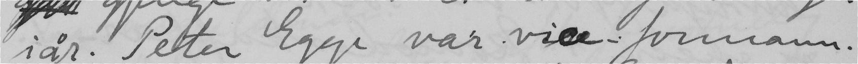iår. Petter Egge var vice-formann.
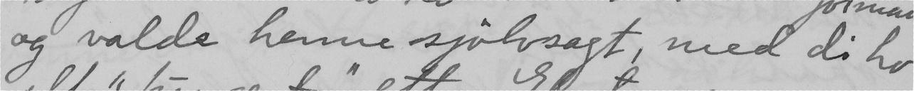og valde henne sjölvsagt, med di ho
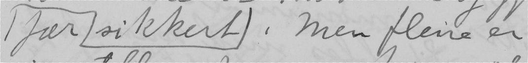fær sikkert. Men fleire er
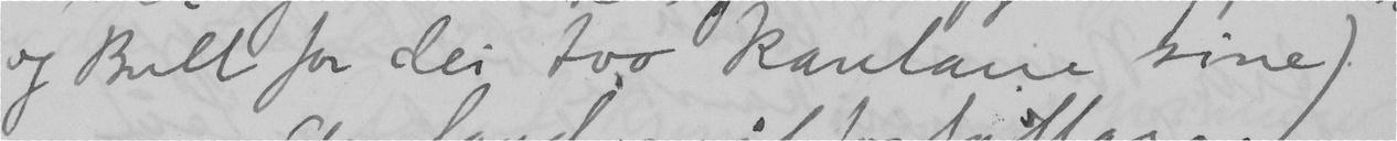og Bull for dei tvo kanlane sine).
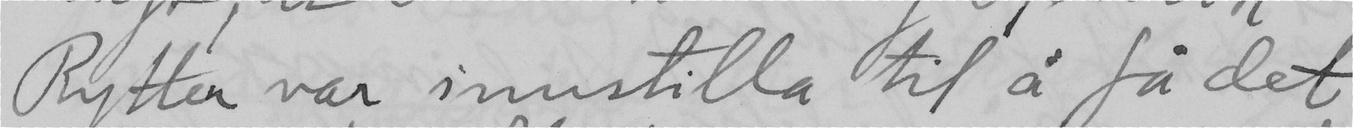Rytter var innstilla til å få det
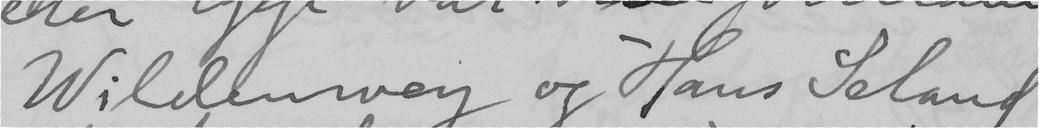Wildenvey og Hans Seland
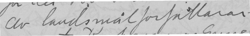Av landsmålforfattarar
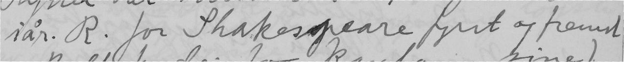iår. R. for Shakespeare fyrst og fremst
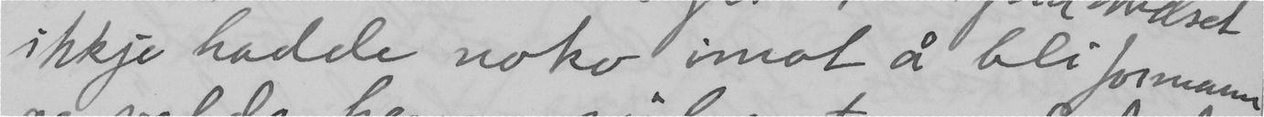ikkje hadde noko imot å bli formann
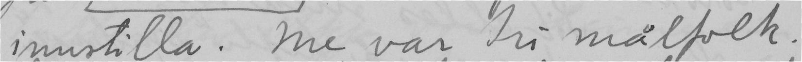innstilla. Me var tri målfolk.
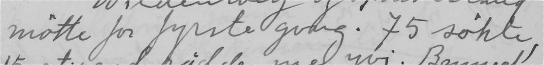mötte for fyrste gang. 75 sökte,
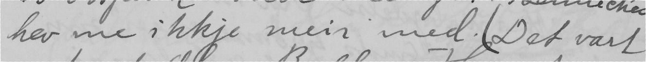hev me ikkje meir med. (Det vart
